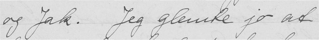og Jak. Jeg glemte jo at
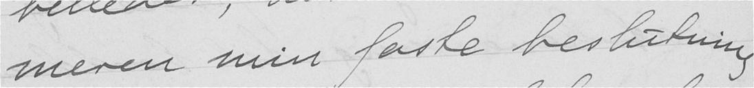meren min faste beslutning
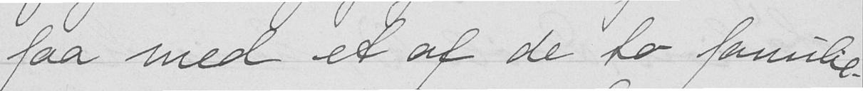faa med et at de to familie
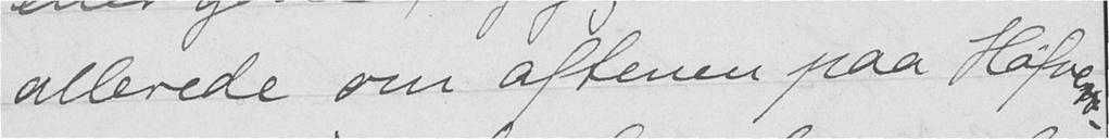allerede om aftenen paa Häfverø
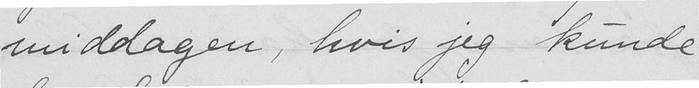middagen, hvis jeg kunde
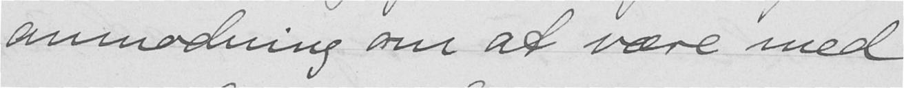ammodning om at være med
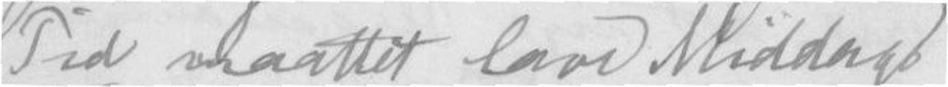Tid maattet lave Middags-
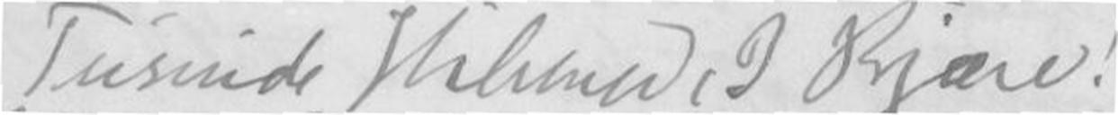Tusinde Hilsener, I Kjære!
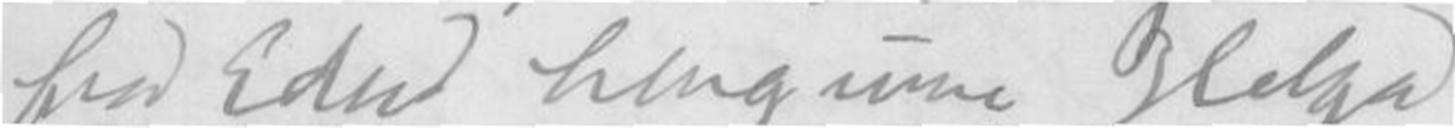fra Eder hengivne Helga
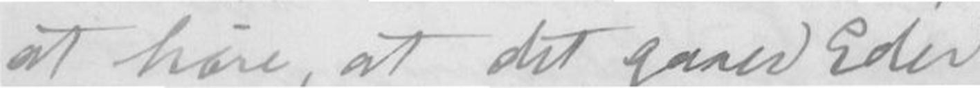at høre, at det gaaer Eder
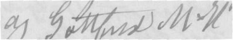og Gottfred M-H
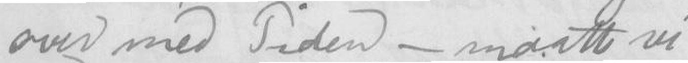over med Tiden - maatte vi
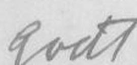godt
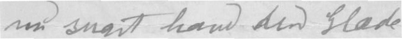nu snart have den Glæde,
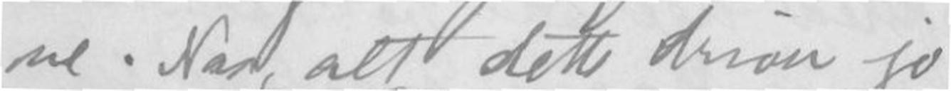ne. Naa, alt dette driver jo
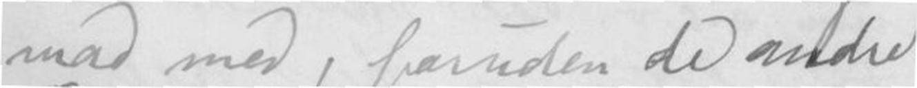mad med, foruden de andre
